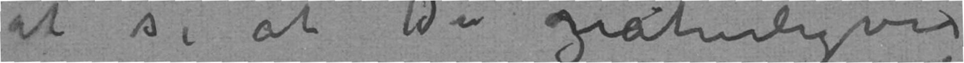at si at Du naturligvis
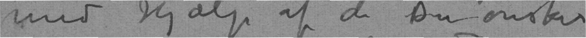med Hjælp af de Du ønsker
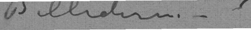Billederne –
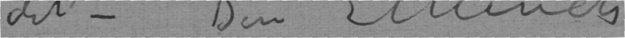det – Din E Munch
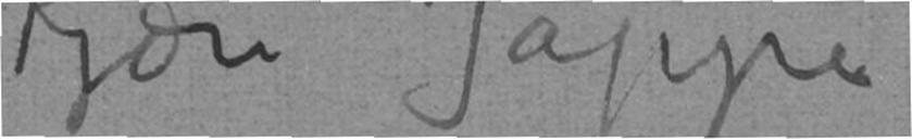Kjære Jappe
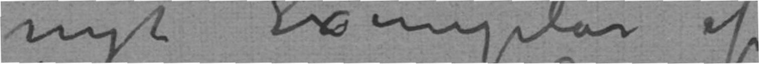nyt Exemplar af
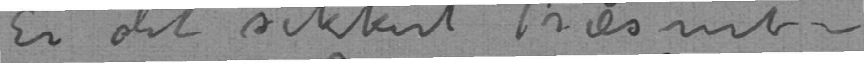Er det sikkert Træsnit –
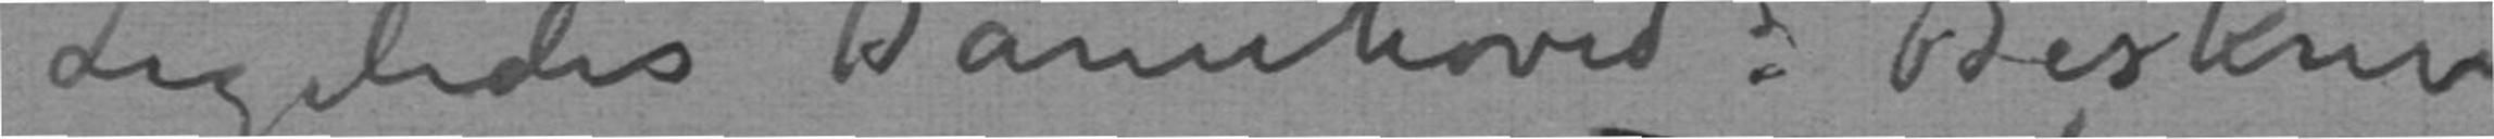Ligeledes Damehoved: Beskriv
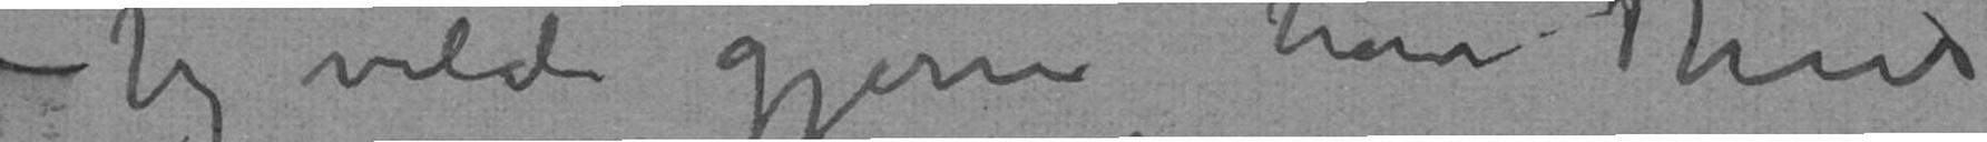– Jeg vilde gjerne have Thiis
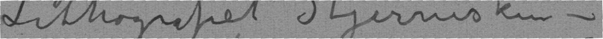Lithografiet Stjerneskin –
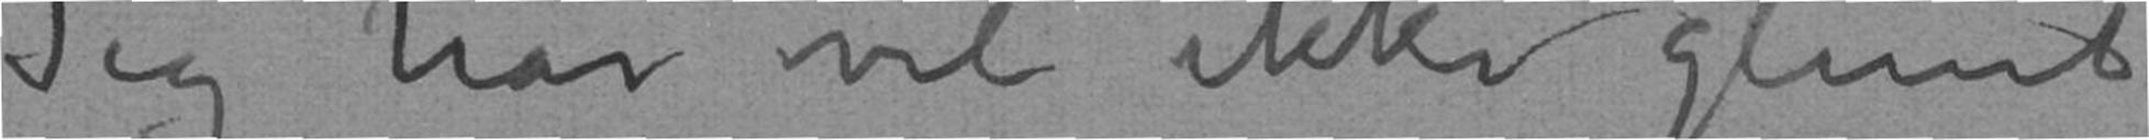Jeg har vel ikke glemt
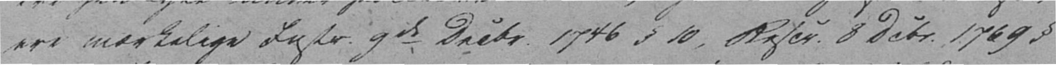ere mærkelige Instr. 9de Decbr. 1746 § 10, Rescr. 8 Dcbr. 1769 §
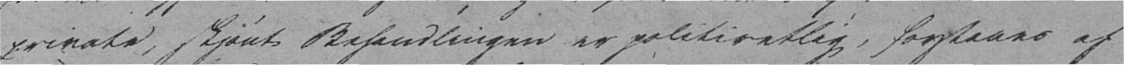private, skjønte Behandlingen er politiretlig, forstaaes af
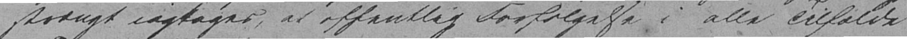strængt iagttages, at offentlig Forfølgelse i alle Tilfælde
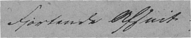Fjortende Afsnit.
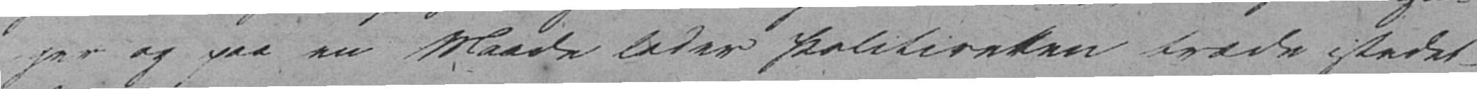ger og paa en Maade lader Politiretten træde istedet-
